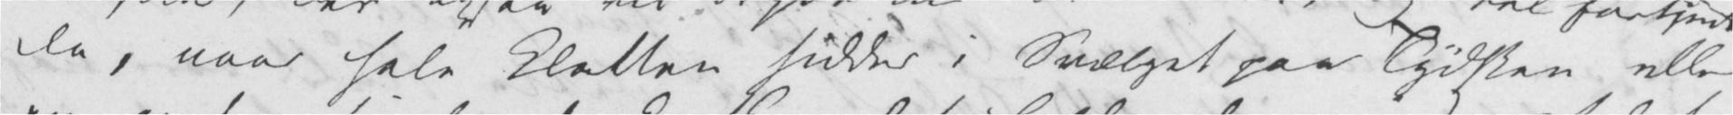Da, naar hele Klatten sidder i Svælget paa Tydsken eller
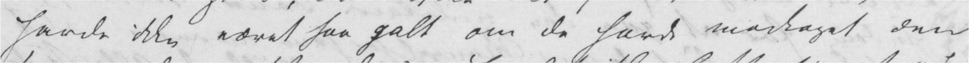havde ikke været saa galt om de havde modtaget den
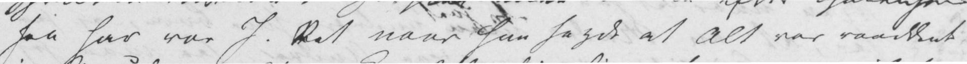saa har vor J. Ret naar han sagde at Alt var raaddent
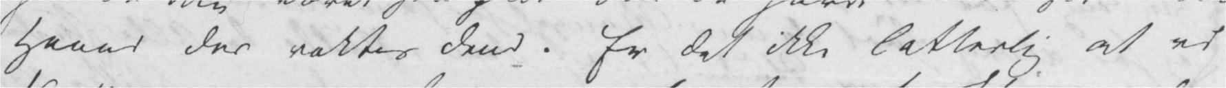Haand der raktes dem. Er det ikke latterlig at vi
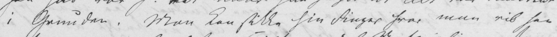i Grunden. Man kan stikke sin Finger hvor man vil saa
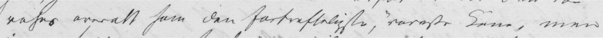roses overalt som den fortreffeligste, «rareste Kone, men
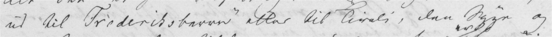ud til Frederiksberre» eller til Tivoli, den Syge og
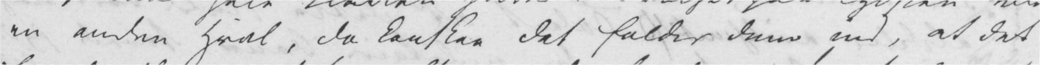en anden Hval, da kanskee det falder dem ind, at det
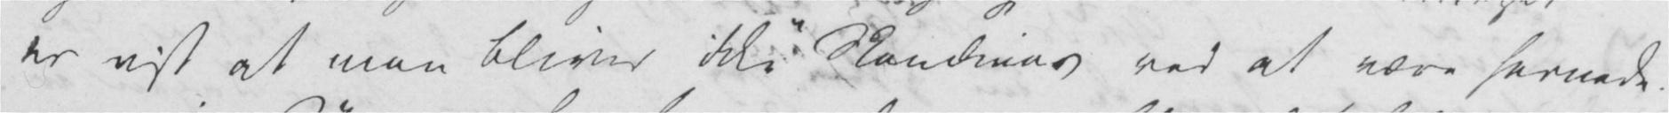er vist at man bliver ikke Skandinav ved at være hernede.
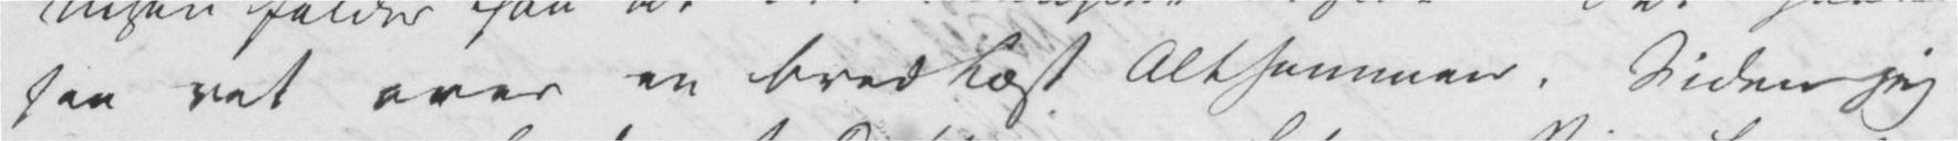saa ret over en bred Læst Altsammen. Siden jeg
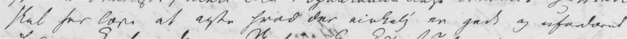skal her lære at agte hvad der virkelig er godt og ufordærvet
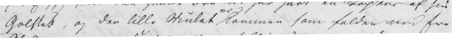Galskab, og den lille Mulat kommen som falden ned fra
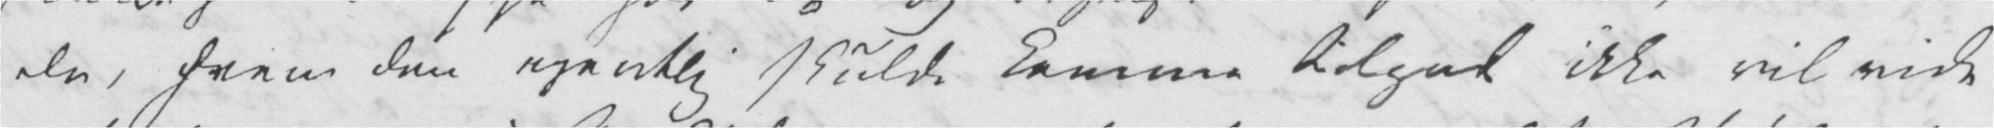de, hvem den egentlig skulde komme tilgode ikke vil vide
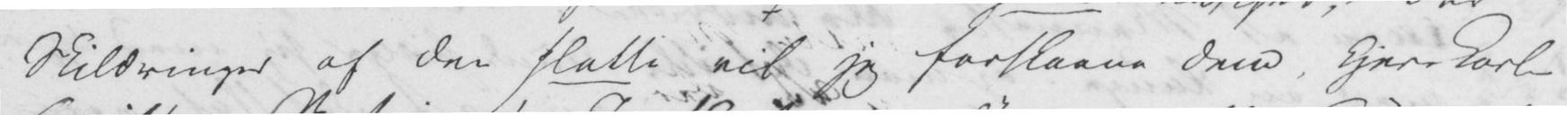Skildringer af den slette vil jeg forskaane Dem, kjere Carl –
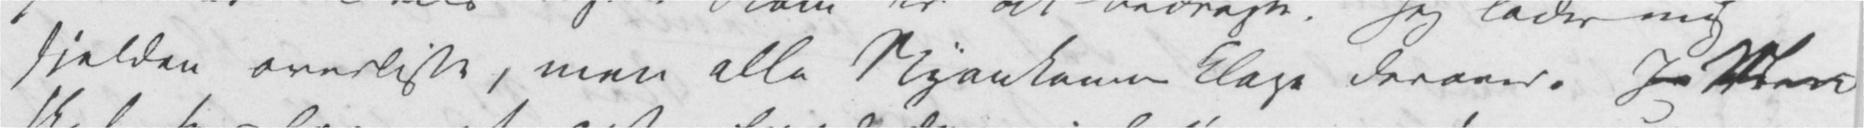sjelden overliste, men alle Nyankomne klage derover. Ja Man
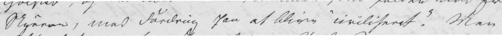Skyerne, med Fordring paa at blive «civiliseret». Men
